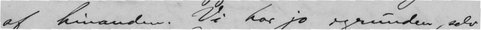af hinanden. Vi har jo igrunden, selv
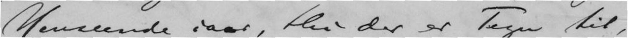Henseende iaar, thi der er Tegn til,
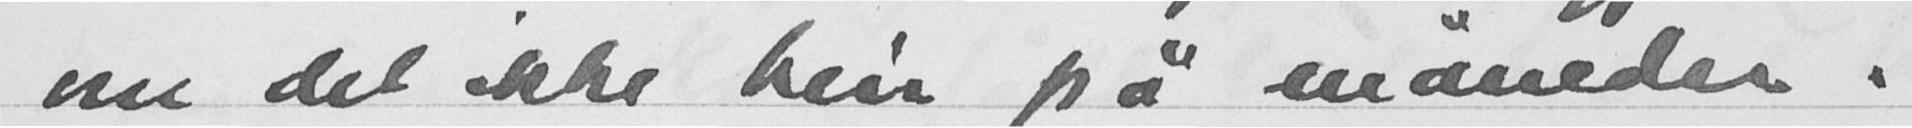om det ikke blir på måneder.
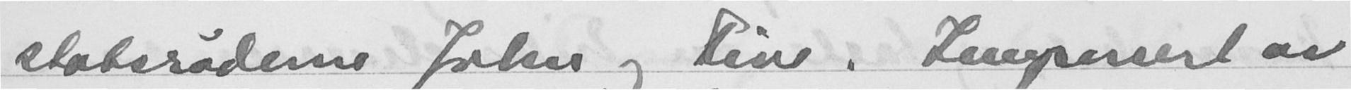statsråderne Johan og Rier . Imponert av
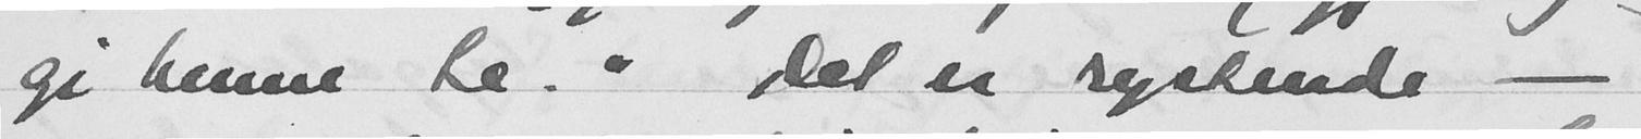gi henne te." Det er rystende -.
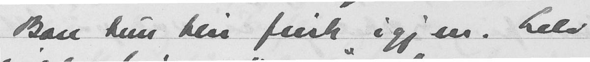Bare hun blir frisk igjen. Selv
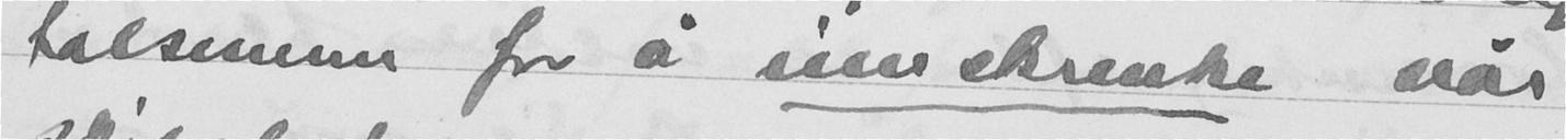talsmenn for å innskrenke vår
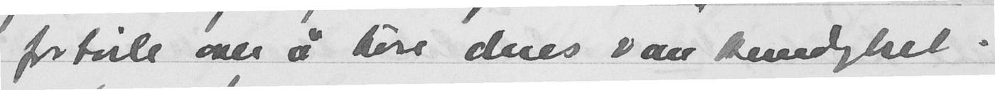fortvile over å høre deres vankundighet"
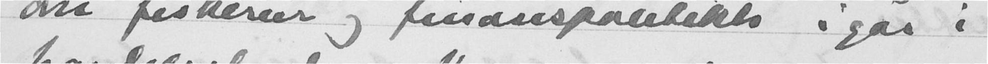om fiskerier og finanspolitikk i går i
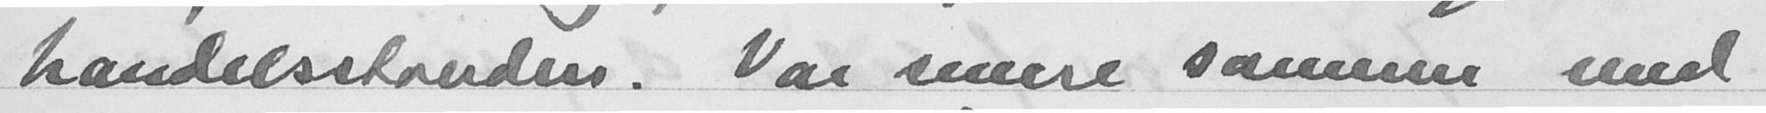handelsstanden. Var senere sammen med
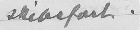skibsfart.
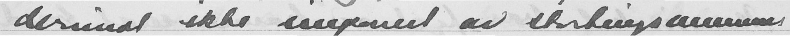derimot ikke imponert av stortingsmannens
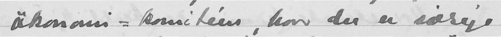økonomi-komitéen, hvor der er søreje
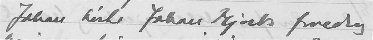Johan hørte Johan Hjorts foredrag
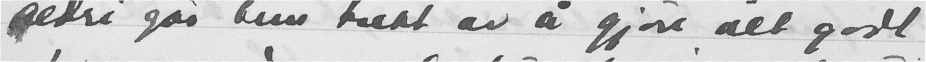aldri går hun trett av å gjøre alt godt,
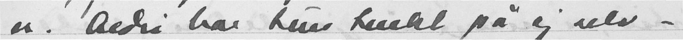er. Aldri har hun tenkt på sig selv -
